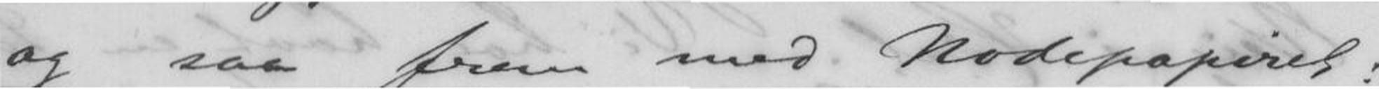og saa frem med Nodepapiret:
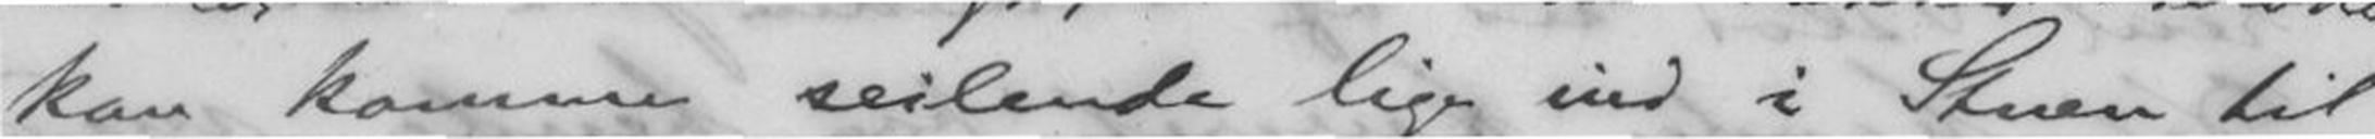kan komme seilende lige ind i Stuen til
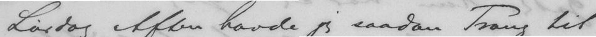Lørdag Aften havde jeg saadan Trang til
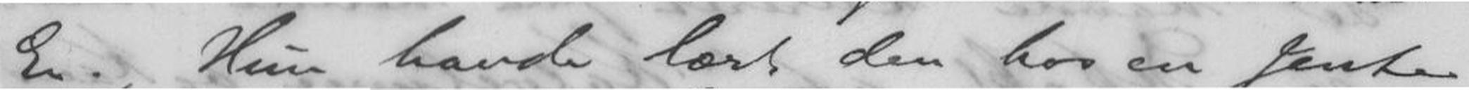En. Hun havde lært den hos en Jente
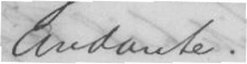Andante.
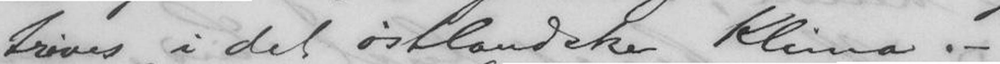trives i det østlandske Klima. -
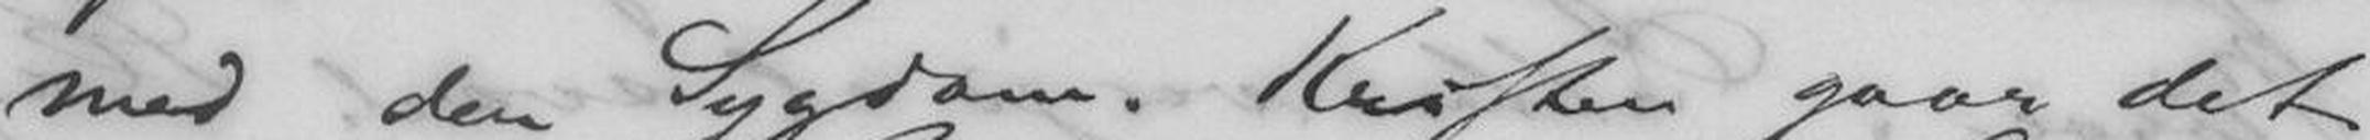med den Sygdom. Kristen gaar det
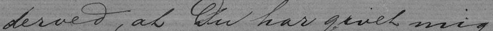derved, at Du har givet mig
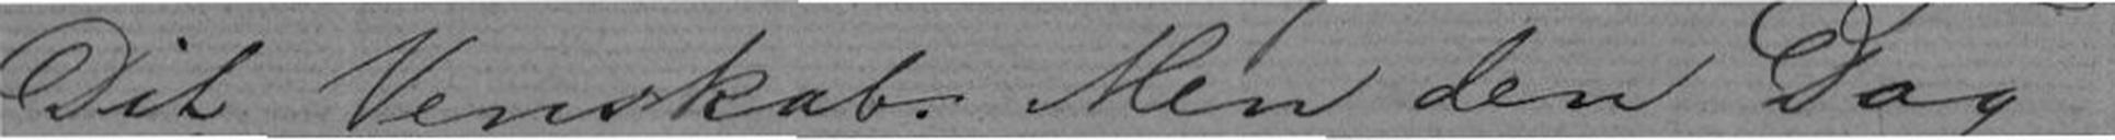Dit Venskab. Man den Dag
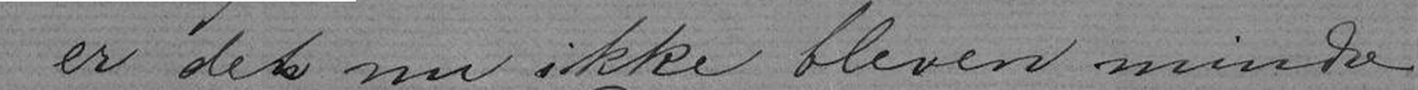er det nu ikke bleven mindre
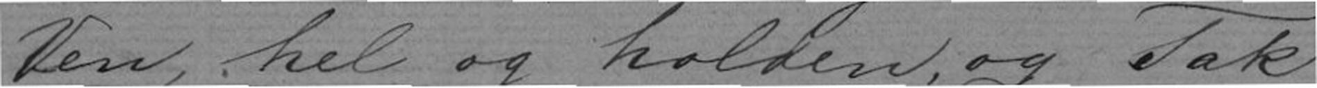Ven, hel og holden, og Tak
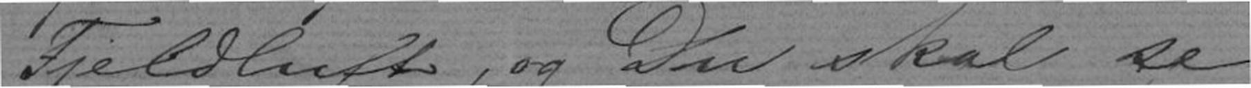Fjeldluft, og Du skal se,
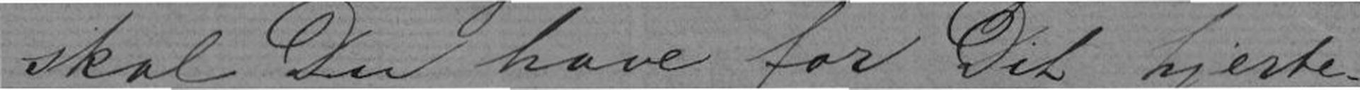skal Du have for Dit hjerte-
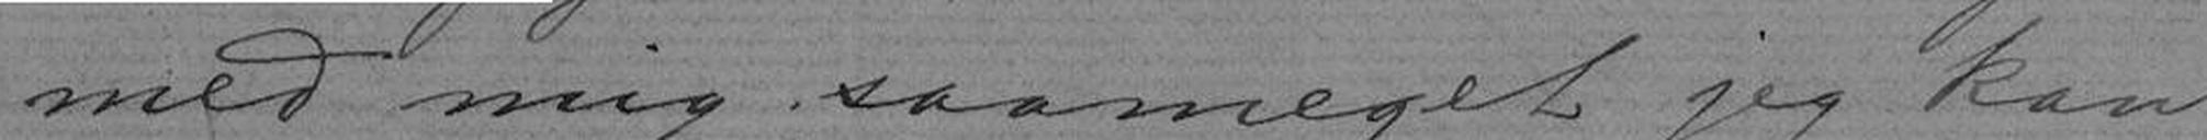med mig saameget jeg kan
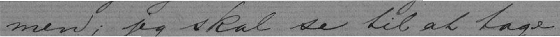men; jeg skal se til at tage
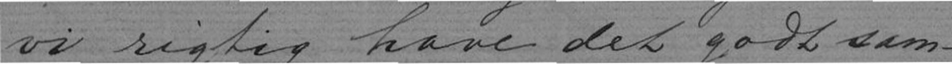vi rigtig have det godt sam-
